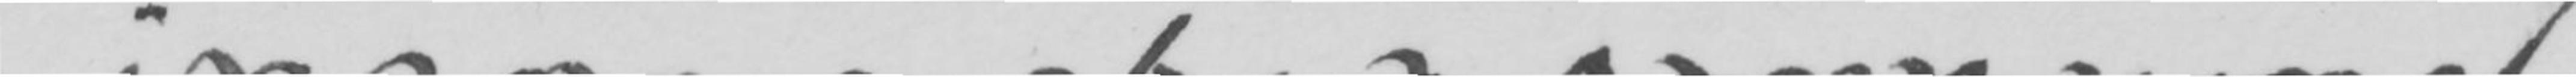presse har d i Kjøbenhavn!
